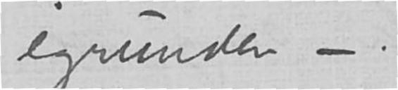igrunden –.
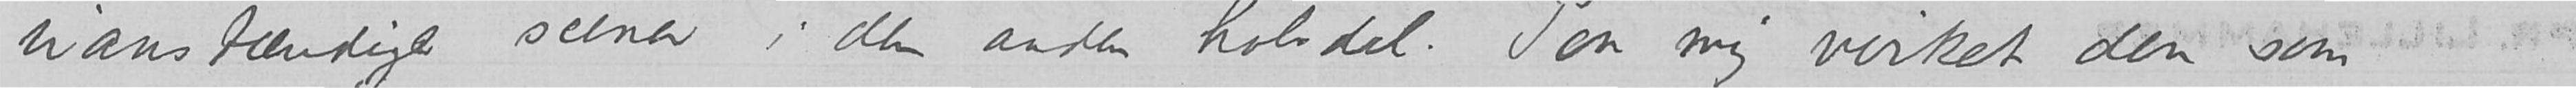uanstændige scener i den anden halvdel. Paa mig virket den som
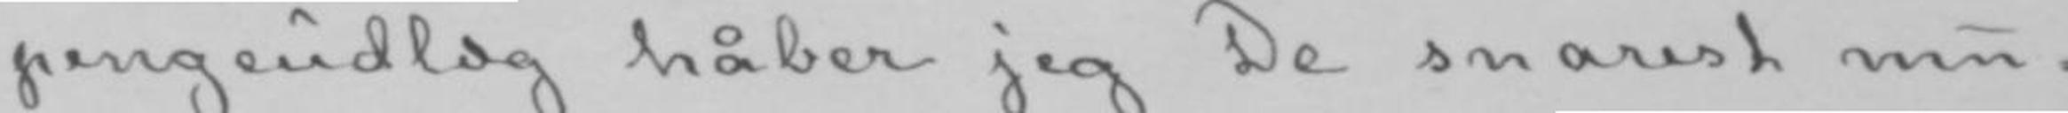pengeudlæg håber jeg De snarest mu-
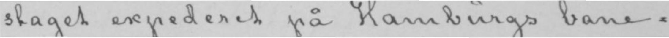staget expederet på Hamburgs bane-
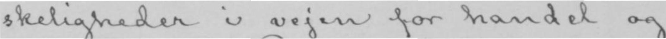skeligheder i vejen for handel og
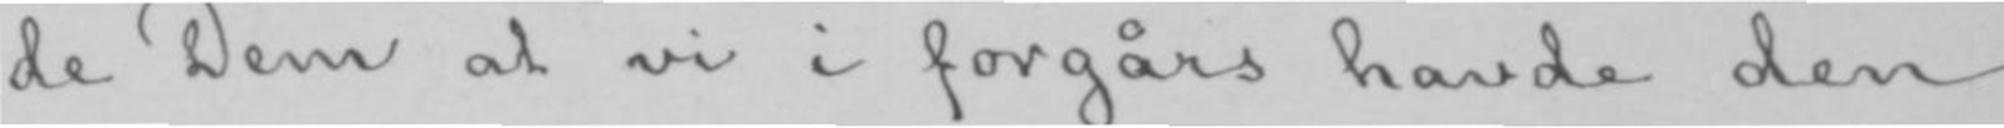de Dem at vi i forgårs havde den
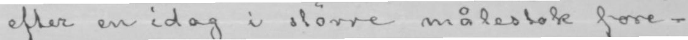efter en idag i større målestok fore-
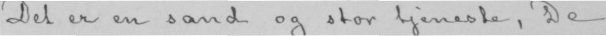Det er en sand og stor tjeneste, De
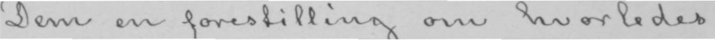Dem en forestilling om hvorledes
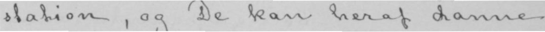station, og De kan heraf danne
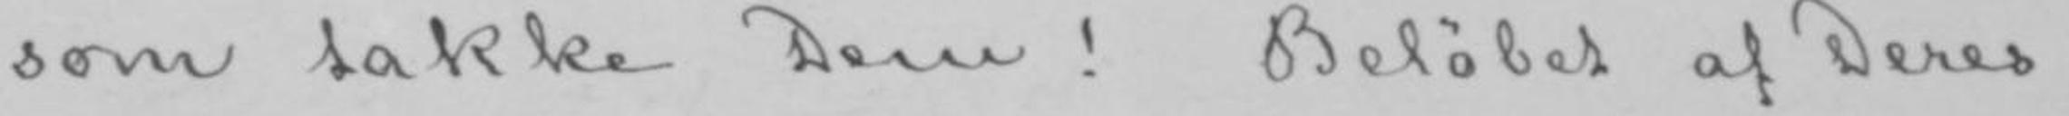som takke Dem! Beløbet af Deres
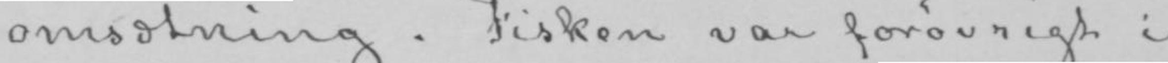omsætning. Fisken var forøvrigt i
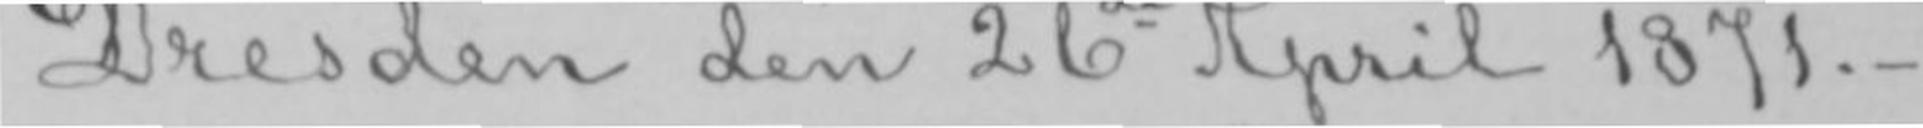Dresden den 26de April 1871.-
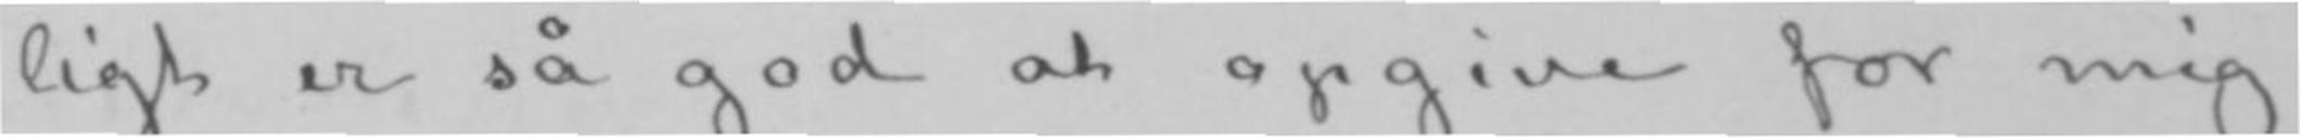ligt er så god at opgive for mig,
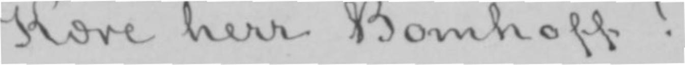Kære herr Bomhoff!
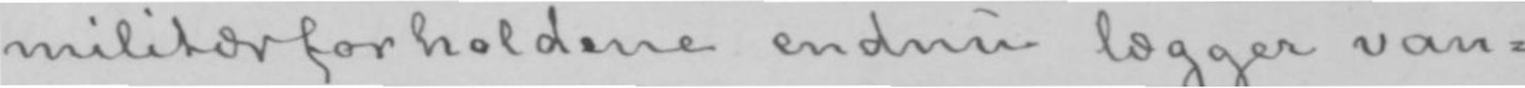militærforholdene endnu lægger van-
